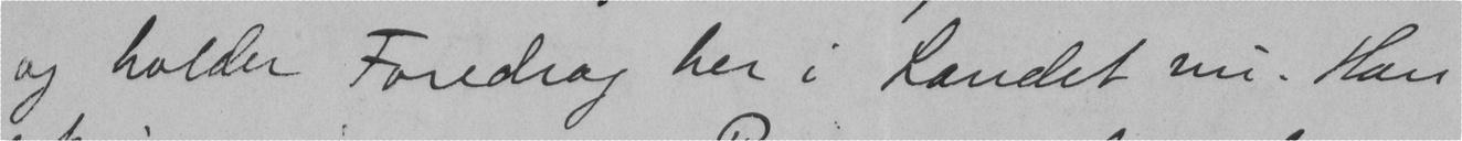og holder Foredrag her i Landet nu. Han
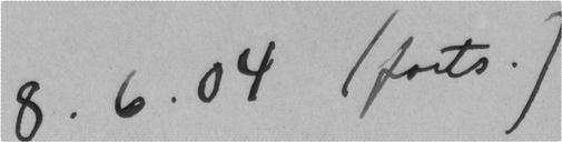8.6.04 (forts.)
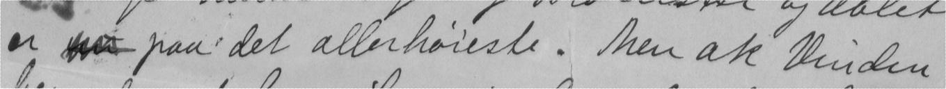er  paa det allerhøieste. Men ak Vinden
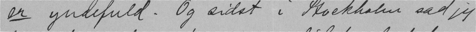er yndefuld. Og sidst i Stockholm sad jeg
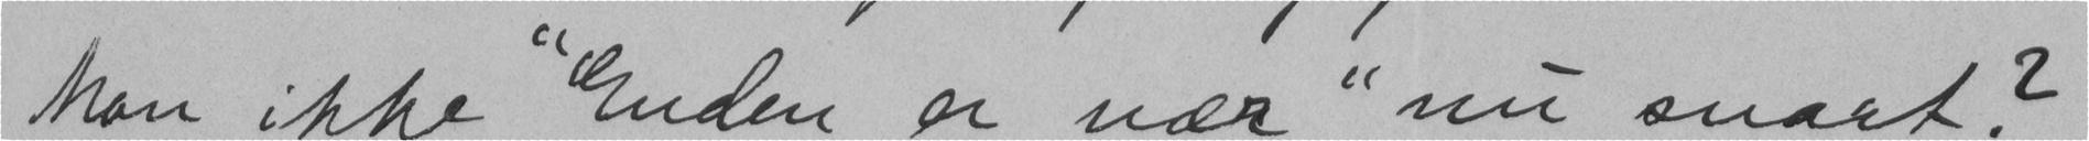Man ikke "Enden er nær " nu snart?
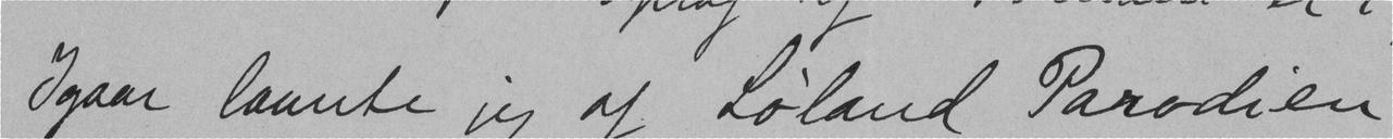Igaar laante jeg af Løland Parodien
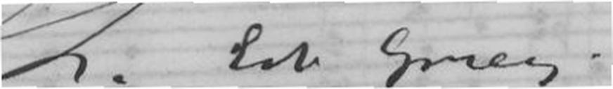Hr. Edv. Grieg.
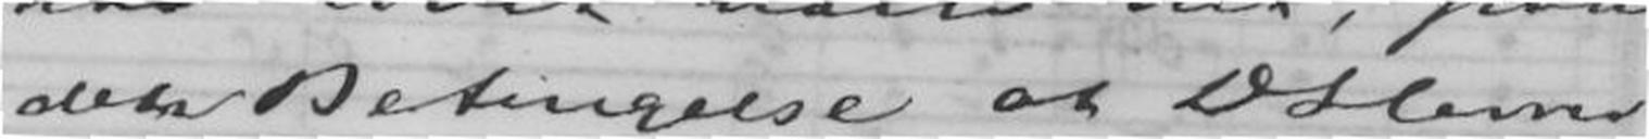den Betingelse at DHerrer
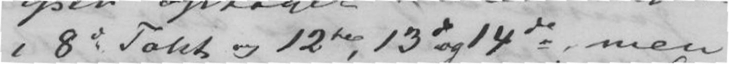i 8de Takt og 12te, 13de og 14de, men
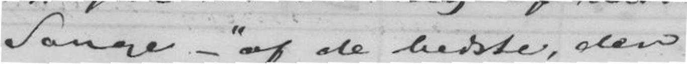Sange - "af de bedste, der
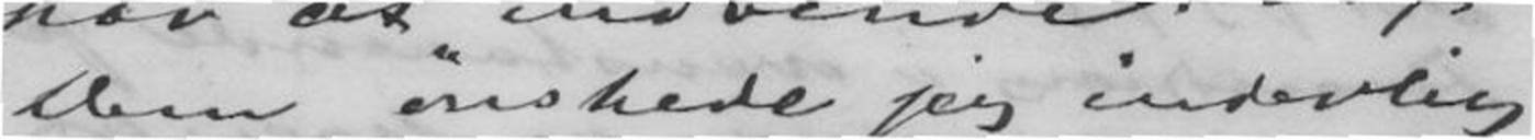Dem ønskede jeg inderlig
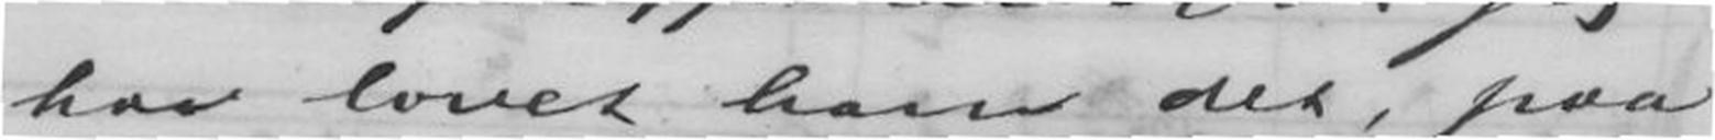har lovet ham det, paa
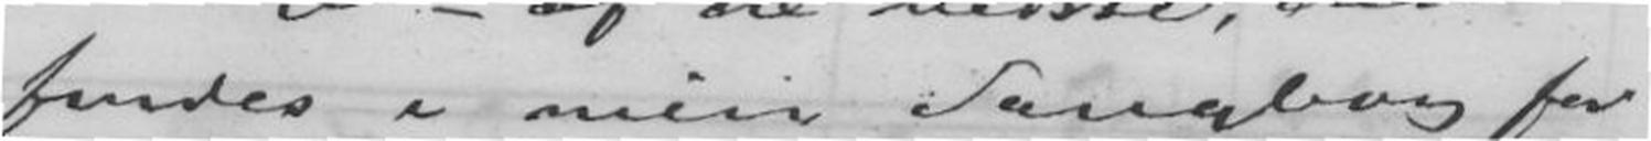findes i min Sangbog for
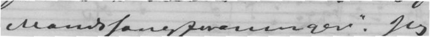Mandssangforeninger". Jeg
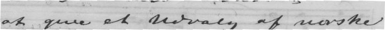at give et Udvalg af norske
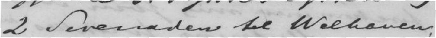2 Serenaden til Welhaven,
